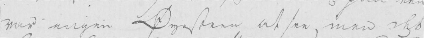var ingen Øyesteen at see, men det
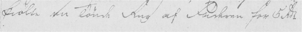kiøbte en Tønde Rug af Faderen for 5 Rdr
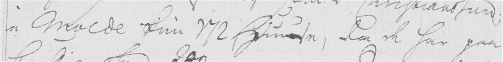i Molde kun 172 Huuse, da de har paa
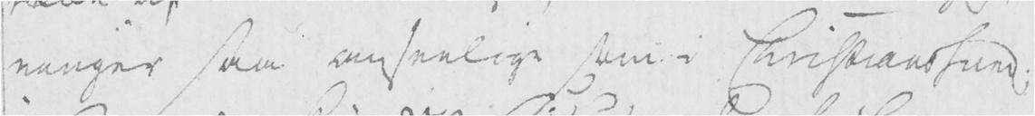ninger saa anseelige som i Christianssund;
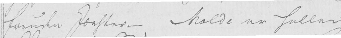foruden Jærster. - Molde er heller
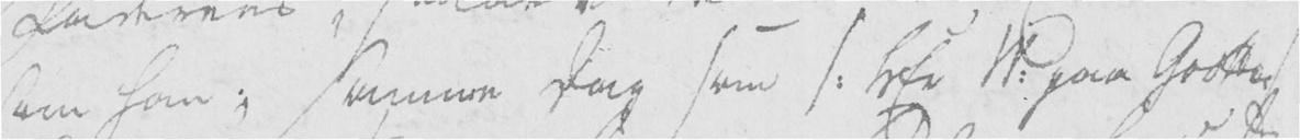som ham; samme dag som /: Hr W. paa Godstad /
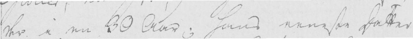der i en 30 Aar; hans eeneste Datter
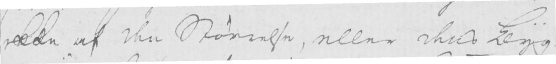ikke af den Størrelse, eller dens Byg-
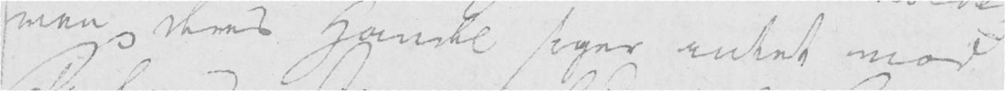men deres Handel siger intet mod
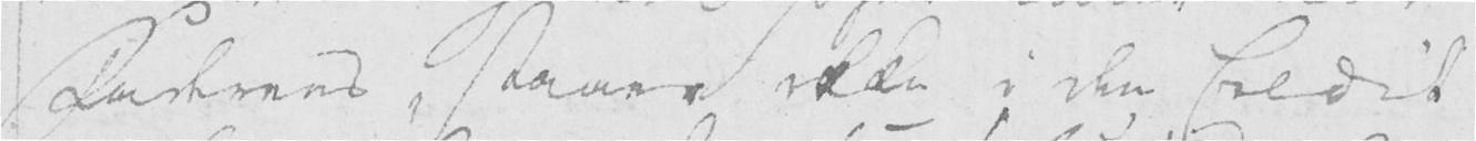Faderens, staaer ikke i den Credit
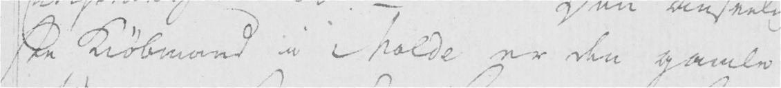ste Kiøbmand i Molde er den gamle
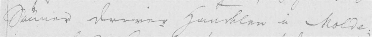Sønner driver Handelen i Molde;
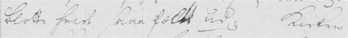blotte hvide saae fældt ud; Kirken
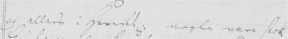og ellers i Hevedet; nogle vare stok
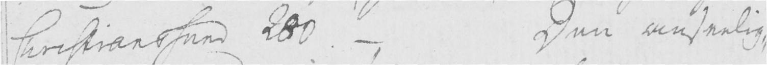Christianssund 280. - Den anseelig-
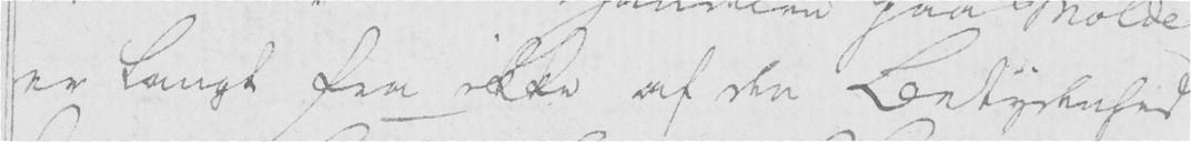er langt fra ikke af den Betydenhed
